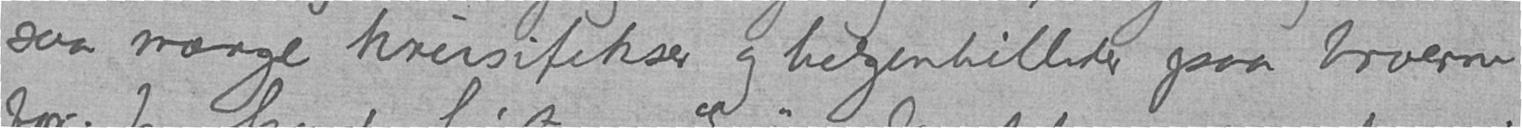saa mange krusifikser og helgenbilleder paa broerne
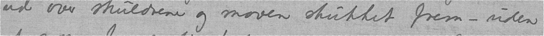ud over skuldrene og maven stukket frem - uden
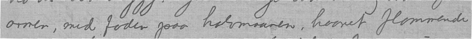armen, med foden paa halvmaanen, haaret flammende
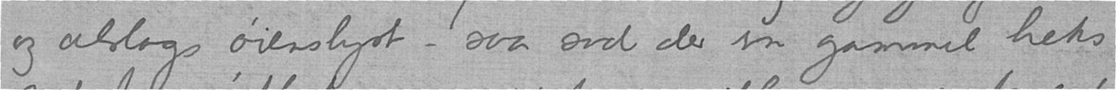og alslags øienslyst - saa sad der en gammel heks
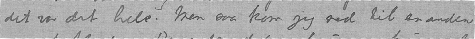det var det hele. Men saa kom jeg ned til en anden
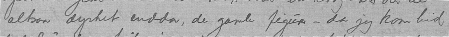altsaa dyrket endda, de gamle figurer - da jeg kom hid
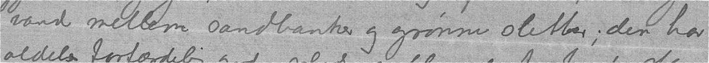vand mellem sandbanker og grønne sletter; den har
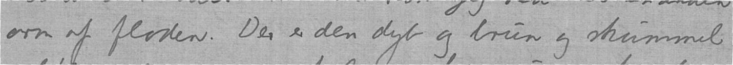arm af floden. Der er den dyb og brun og skummel
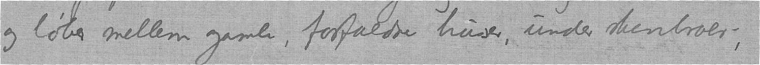og løber mellem gamle, forfaldne huser, under stenbroer-
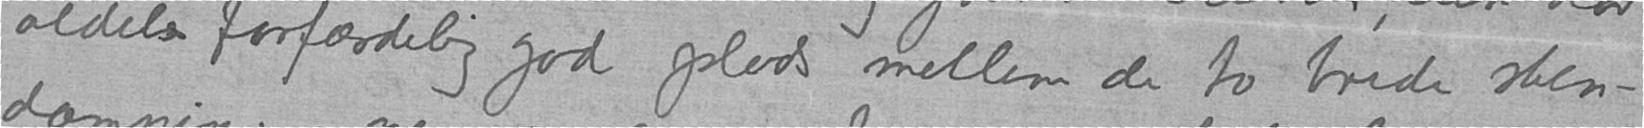aldeles forfærdelig god plads mellem de to brede sten-
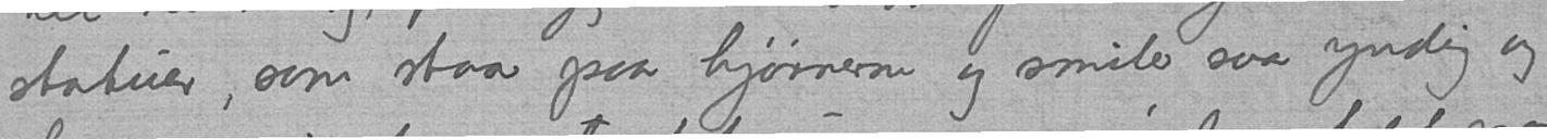statuer, som staar paa hjørnerne og smiler saa yndig og
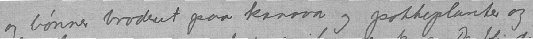og bønner brodert paa kanava og potteplanter og
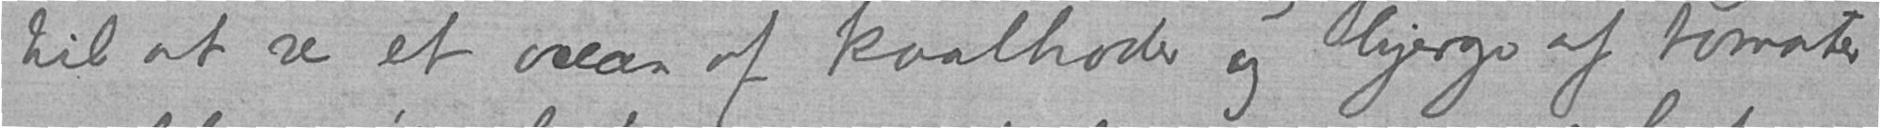til at se et ocean af kaalhoder og bjerge af tomater
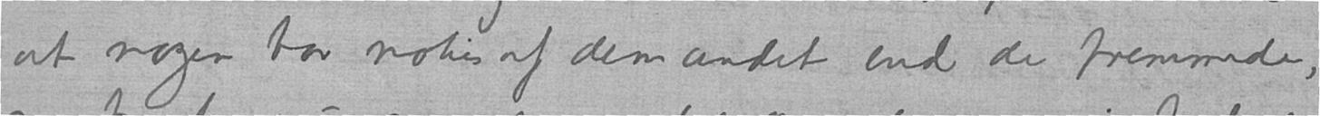at nogen tar notis af den andet end de fremmede,
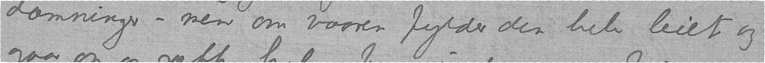dæmninger - men om vaaren fylder den hele leiet og
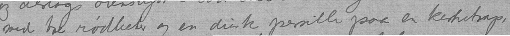med tre rødbeter og en dusk persille paa en kirketrap,
